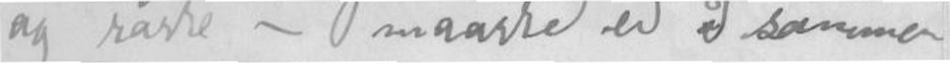og raske - maaske er I sammen
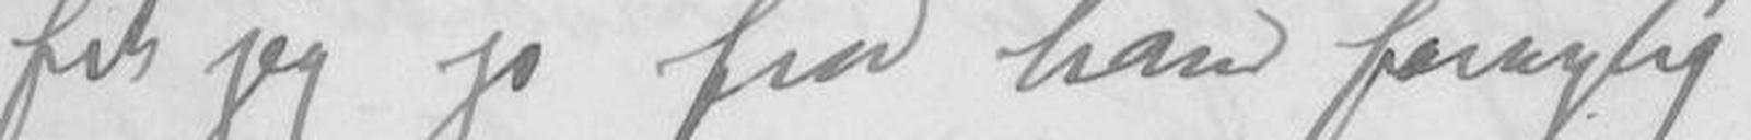fikk jeg jo fra ham fornylig.
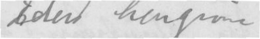Eders hengivne
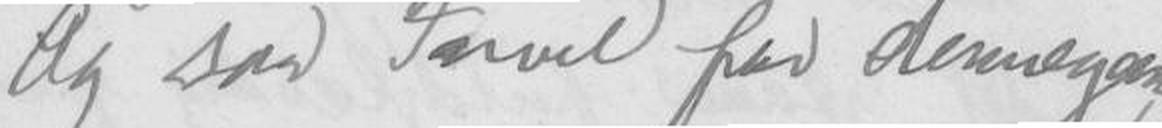Og saa Farvel for dennegang,
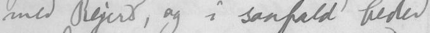med Beyers, og i saafald beder
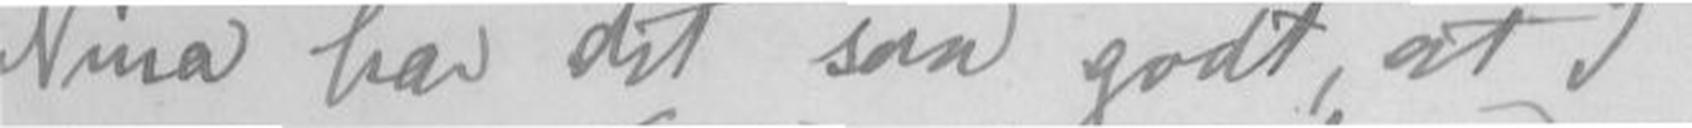Nina har det saa godt, at I
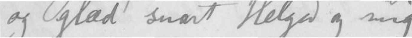og glæd snart Helga og mig
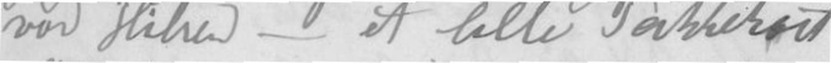vor Hilsen - et lille Takkekort
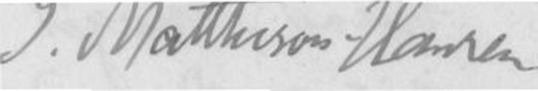G. Matthison=Hansen
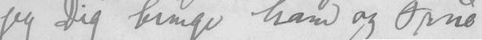jeg Dig bringe ham og Frue
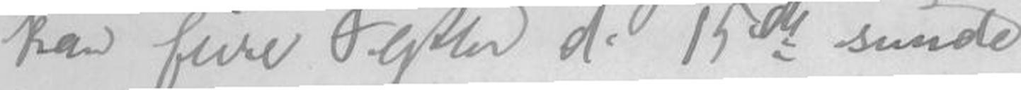kan feirer Festen d. 15de sunde
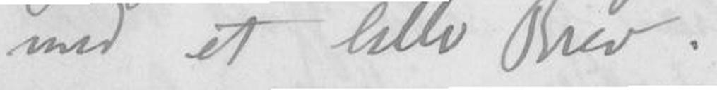med et lille Brev.
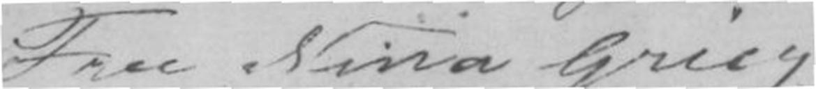Fru Nina Grieg.
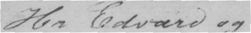Hr Edvard og
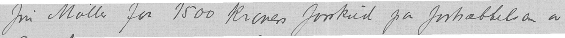fru Møller faa 1500 kroners forskud paa fortsættelsen av
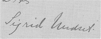Sigrid Undset.
